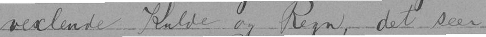vexlende Kulde og Regn, det seer
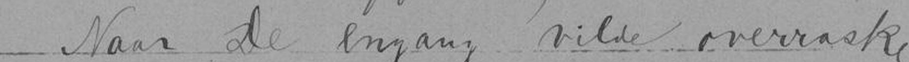Naar De engang vilde overraske
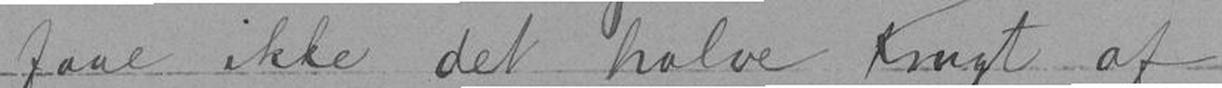faae ikke det halve Frugt af
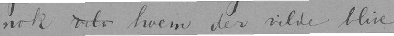nok vil hvem der vilde blive
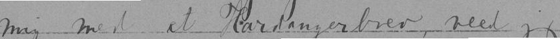mig med et Hardangerbrev, ved jeg
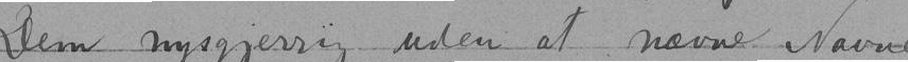Dem nysgjerrig uden at nævne Navne,
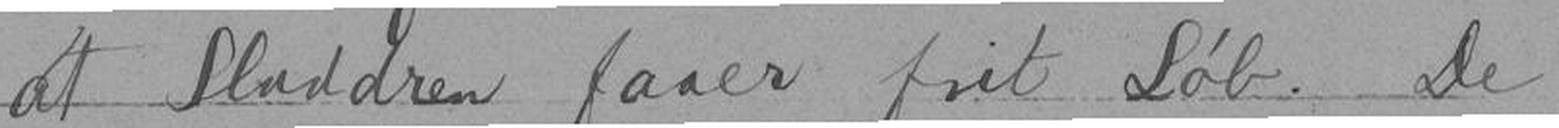at Sladdren faaer frit Løb. De
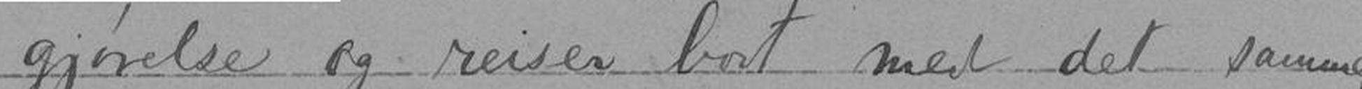gjørelse og reiser bort med det samme,
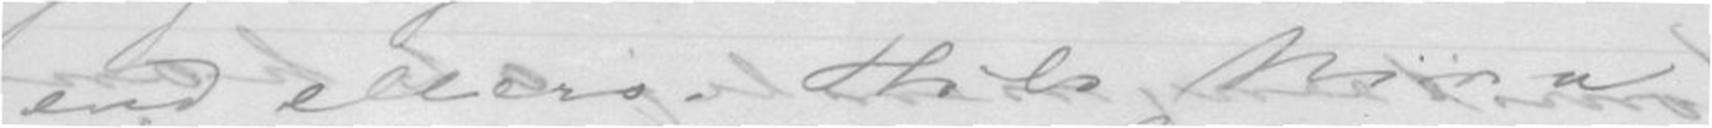end ellers. Hils Nina
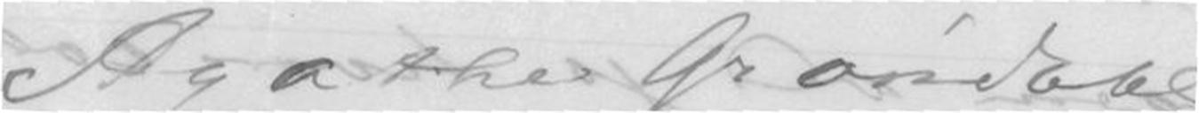Agathe Grøndahl
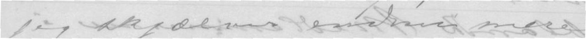jeg skjælver endnu mere
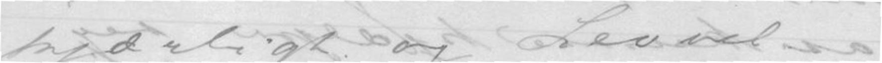kjærligt og Lev vel
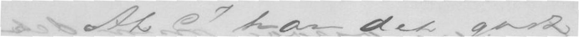At I har det godt
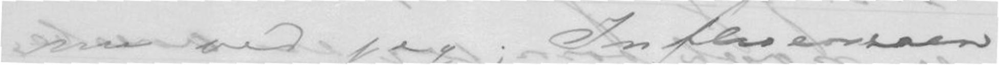nu ved jeg; Influensaen
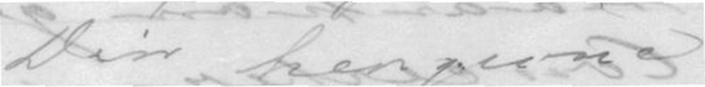Din hengivne
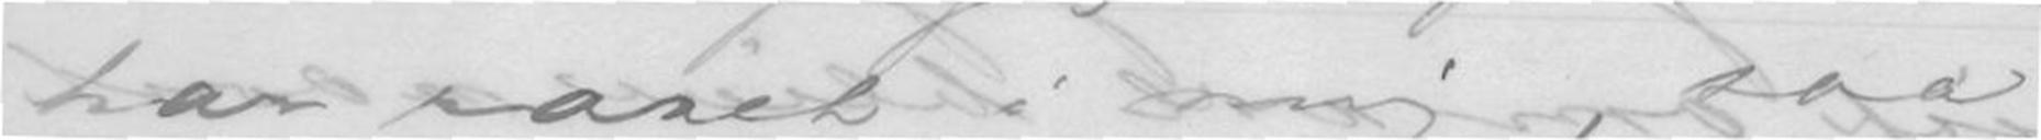har raset i mig, saa
